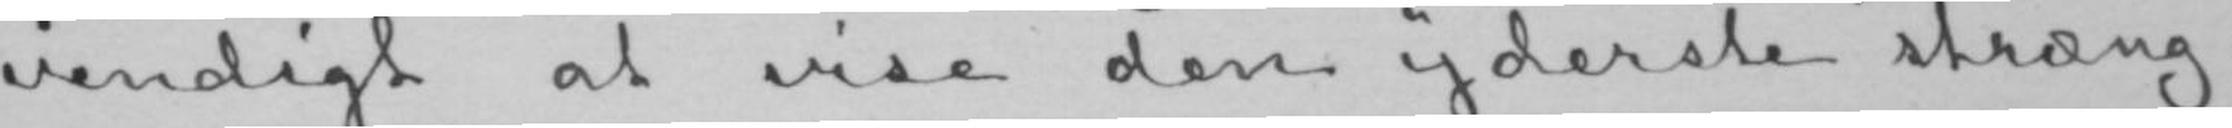vendigt at vise den yderste stræng-
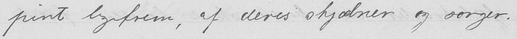pint ligefrem, af deres skjæbner og sorger.
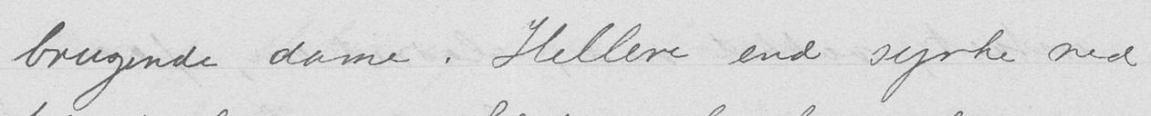brugende dame. Hellere end at synke ned
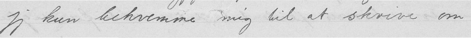jeg kun bekvemme mig til at skrive om
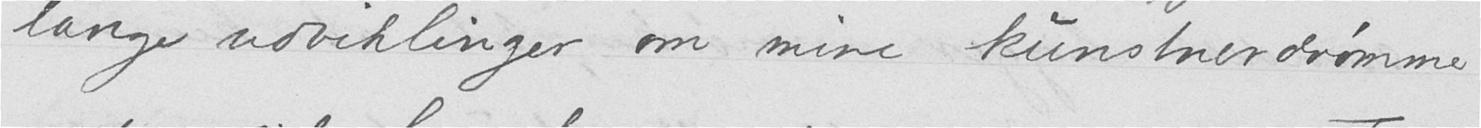lange udviklinger om mine kunstnerdrømme
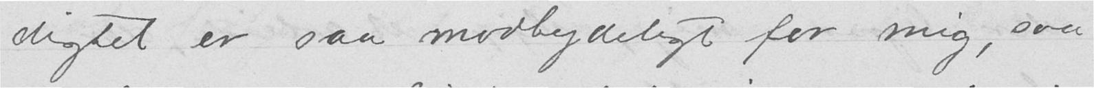digtet er saa modbydeligt for mig, saa
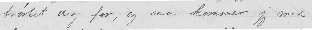trøstet dig for, og saa kommer jeg med
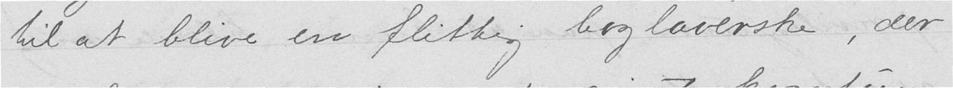til at blive en flittig boglaverske, der
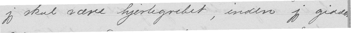jeg skal være hjertegrebet, inden jeg gidder
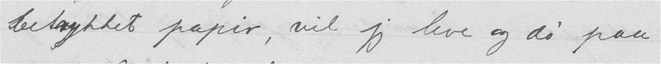betrykket papir, vil jeg leve og dø på
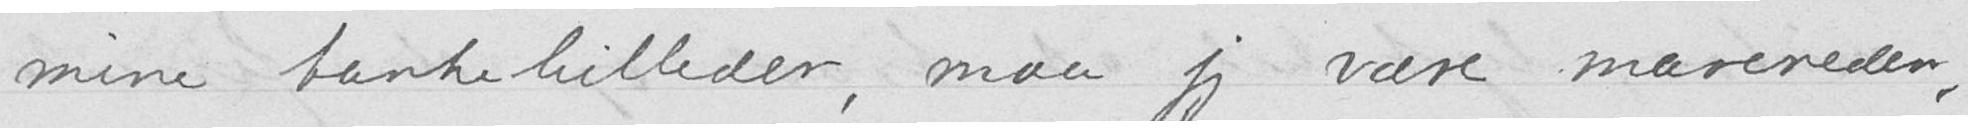mine tankebilleder, må jeg være marereden,
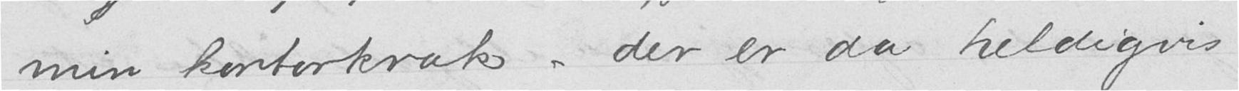min kontorkrak - der er da heldigvis
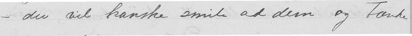- du vil kanske smile ad dem og tænke
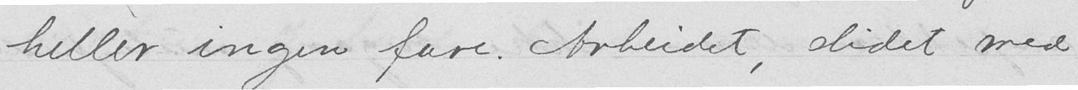heller ingen fare. Arbeidet, slidet med
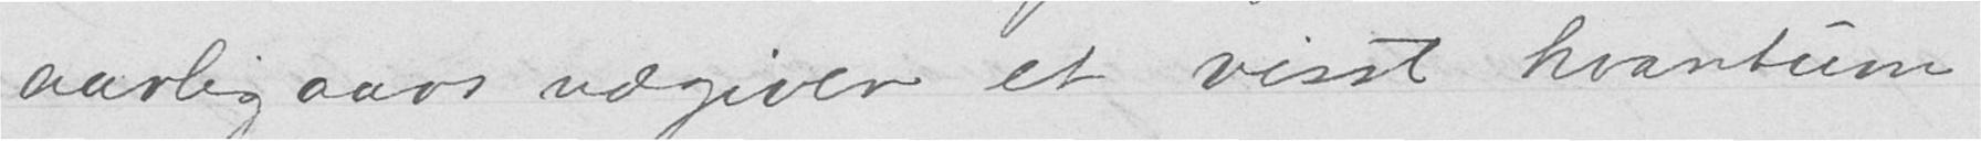aarligaars udgiver et visst kvantum
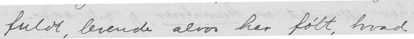fuldt, levende alvor har følt, hvad
